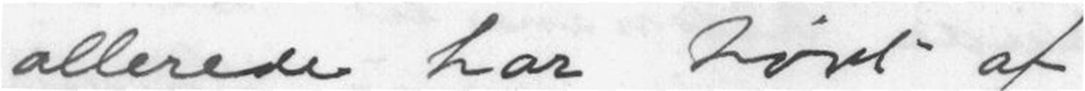allerede har hørt af
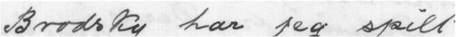Brodsky har jeg spilt
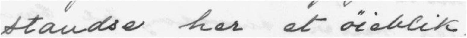standse her et øieblik.
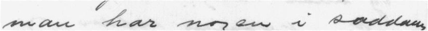man har nogen i saadanne
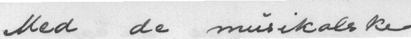Med de musikalske
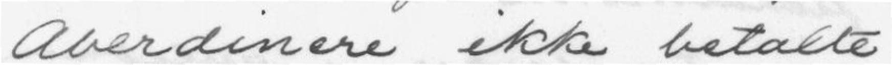Aberdinere ikke betalte
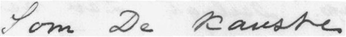Som De kanske
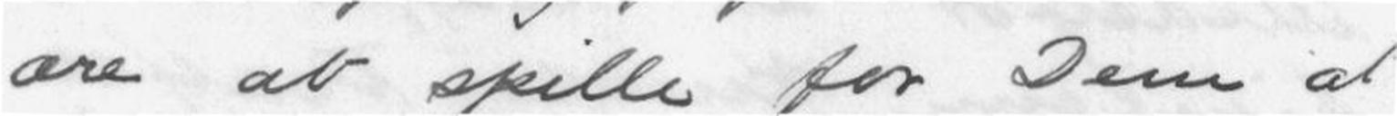ære at spille for Dem at
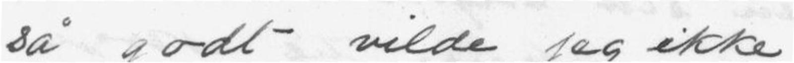så godt vilde jeg ikke
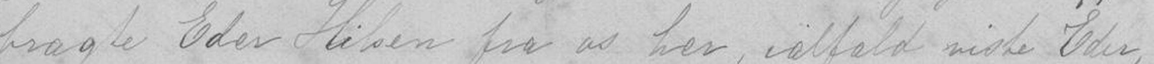brakte Eder Hilsen fra os her, valfald miste Eder,
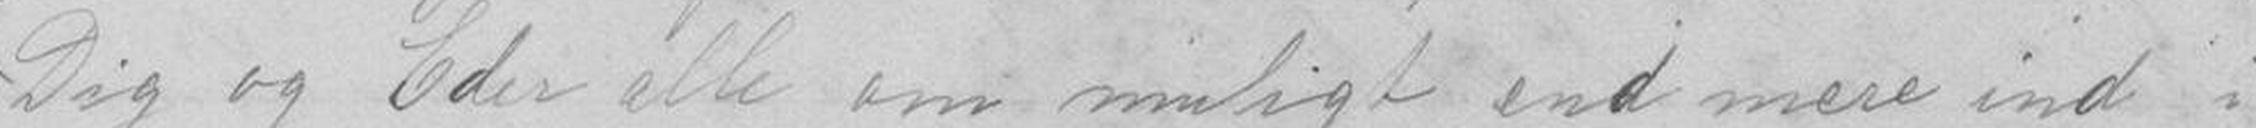Dig og Eder alle om muligt end mere ind i
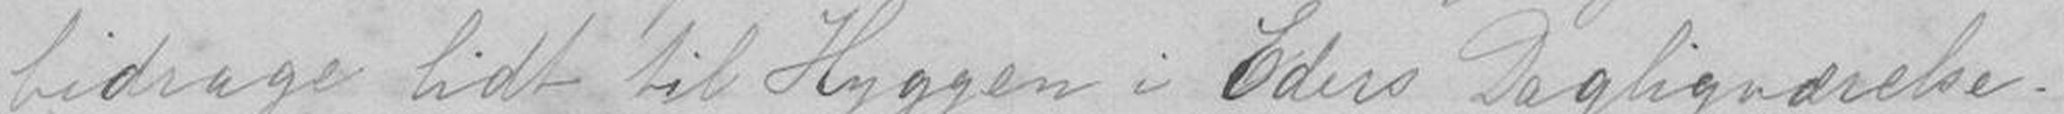bidrage lidt til Hyggen i Eders Dagligværelse.
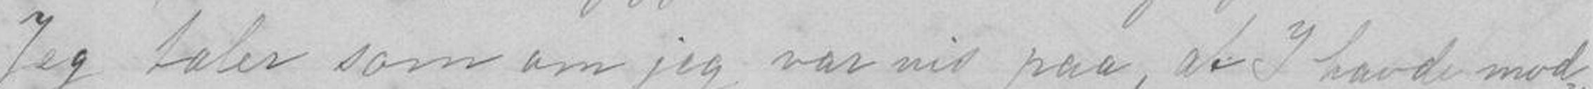Jeg taler som om jeg var vis paa, at I havde mod-
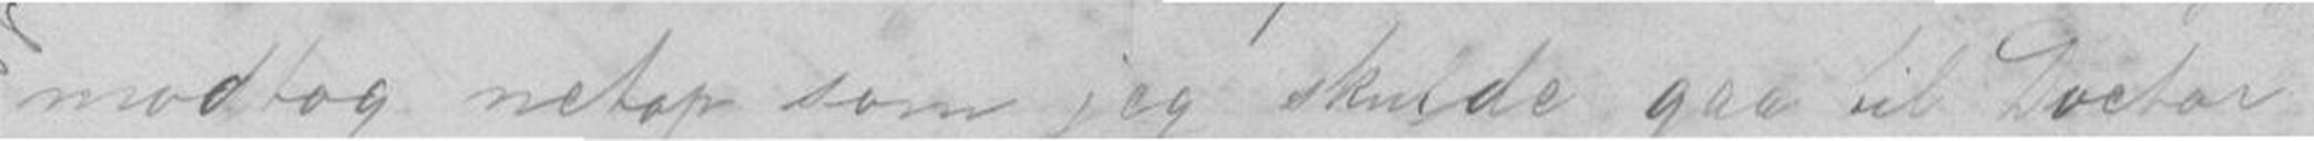modtog netop som jeg skulde gaa til Doctor
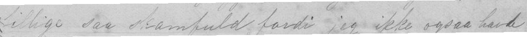tilbige saa skamfuld fordi jeg ikke også havde
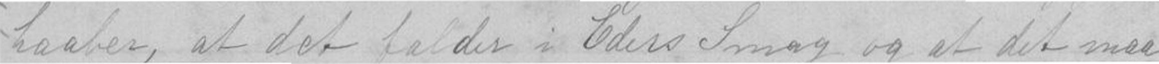haaber, at det falder i Eders Smag og at det maa
